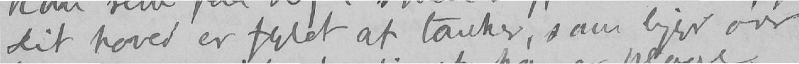dit hoved er fyldt af tanker, som ligger over
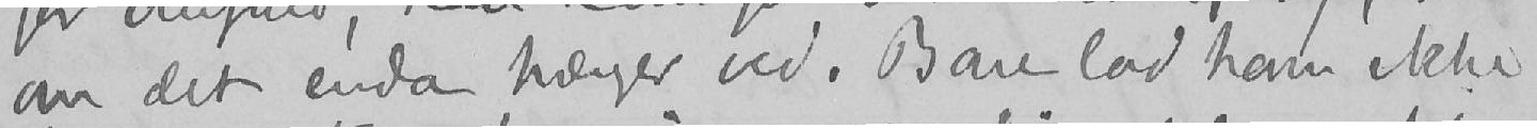om det enda hænger ved. Bare lad ham ikke
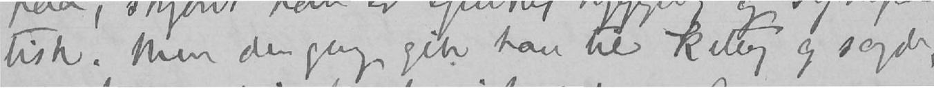tisk. Men dengang gik han til Kitty og sagde,
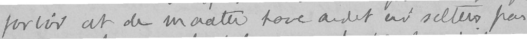forbød at de maatte have andet end selters paa
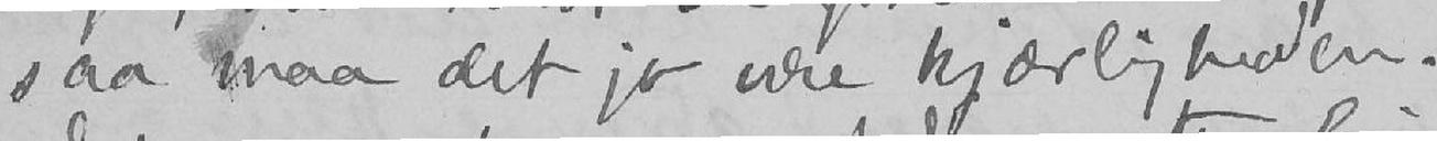saa maa det jo være kjærligheden.
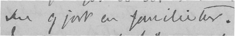Du gjort en familietur.
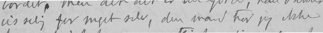visselig for meget selv, den mand tror jeg ikke
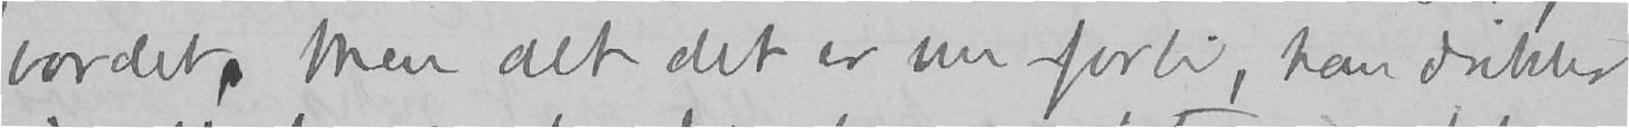bordet. Men alt det er nu forbi, han drikker
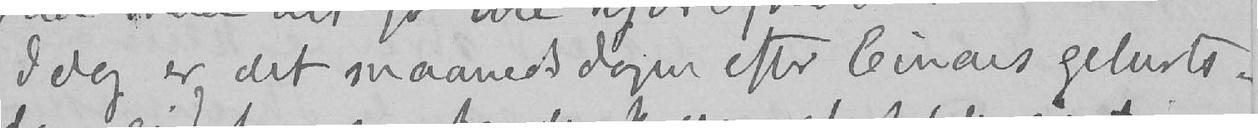Idag er det maanedsdagen efter Einars geburts-
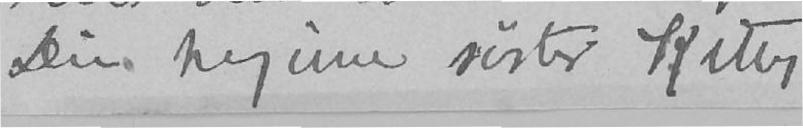Din hengivne søster Kitty.
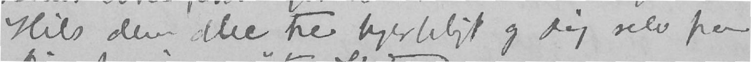Hils dem alle tre hjerteligt og dig selv fra
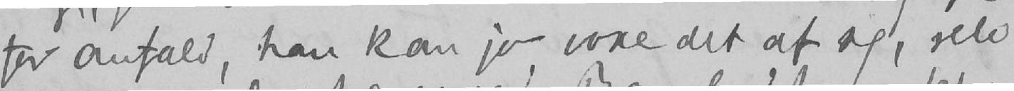for anfald, han kan jo voxe det af sig, selv
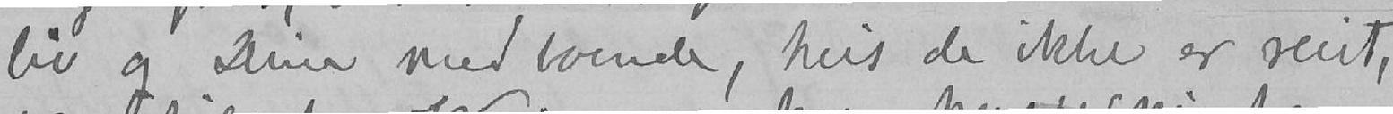liv og Dine medboende, hvis de ikke er reist,
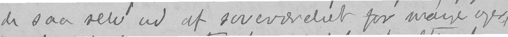de saa selv ud af soveværelset for mange uger,
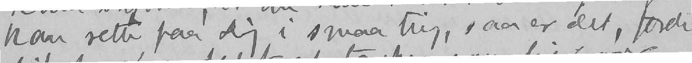kan rette paa Dig i smaa ting, saa er det, fordi
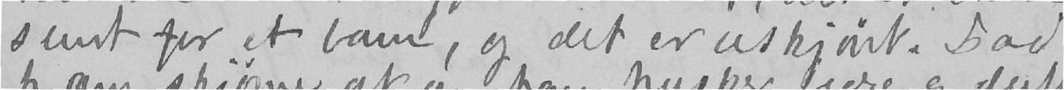sundt for et barn, og det er uskjønt. Lad
